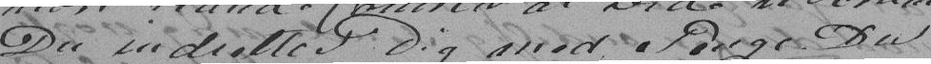Du indrettet Dig med Penge. Du
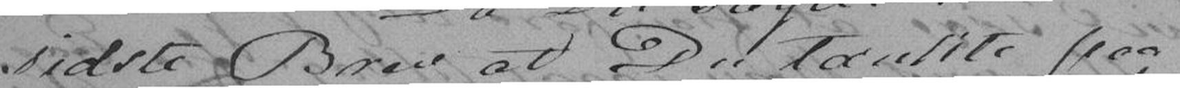sidste Brev at Du tænkte paa
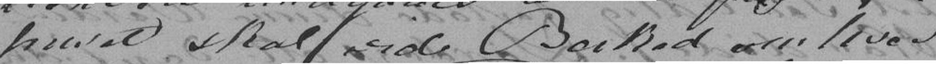huset skal vide Besked om hver
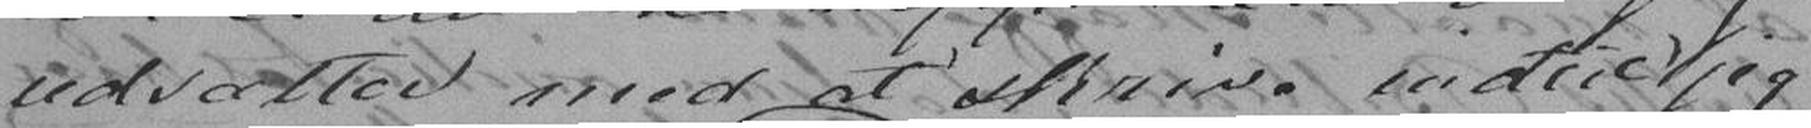udsætter med at skrive indtil jeg
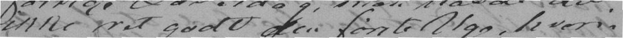ikke ret godt den først Uge, hvori-
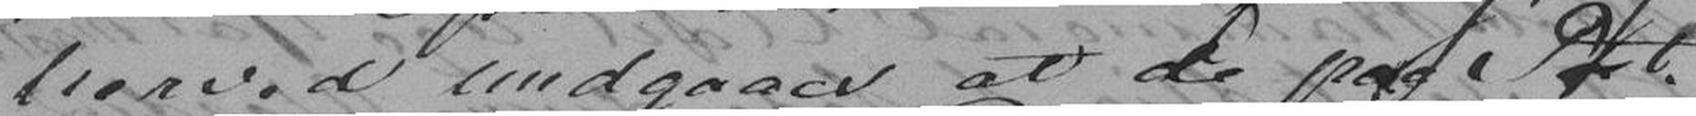herved undgaaes at de paa Post-
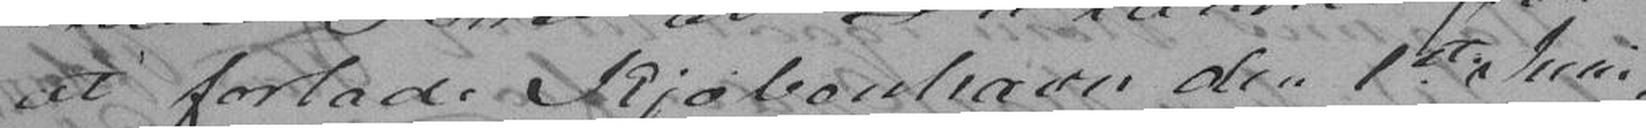at forlade Kjøbenhavn den 1ste Juni,
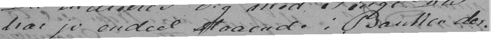har jo endeel staaende i Banken der-
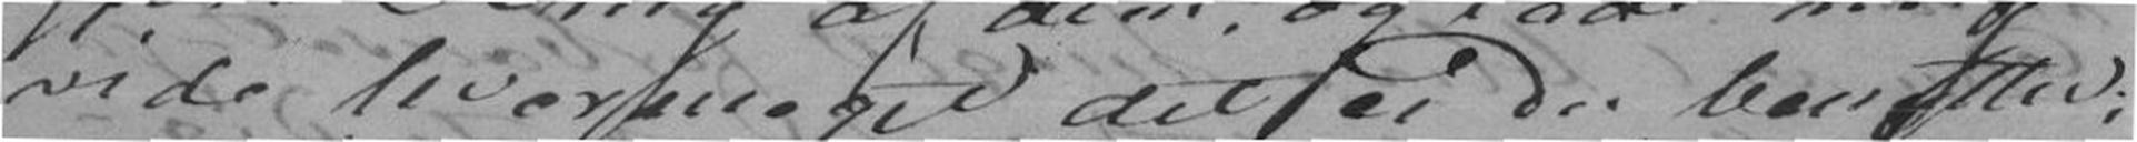vide hvormeget det er Du benytter;
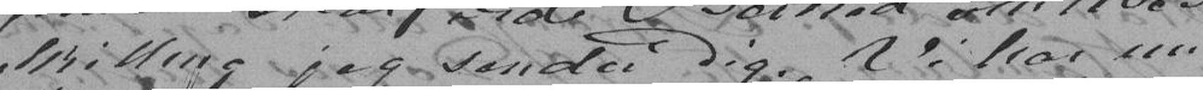Shilling jeg sender Dig. Vi har nu
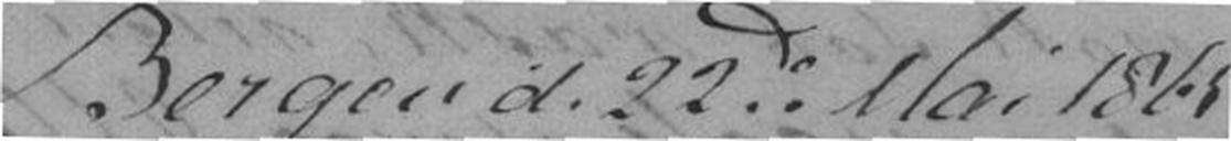Bergen d. 22de Mai 1865.
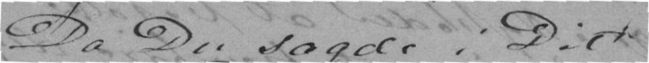Da Du sagde i Dit
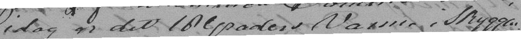idag er det 18 Graders Varme i Skyggen.
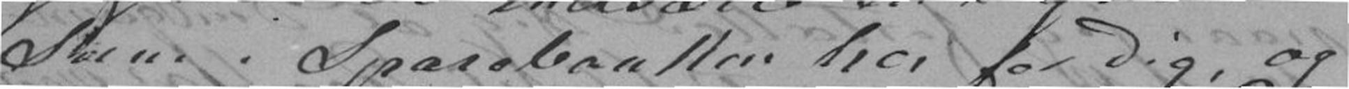Sum i Sparebanken her for Dig, og
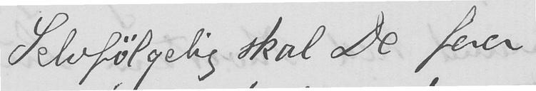Selvfølgelig skal De faa
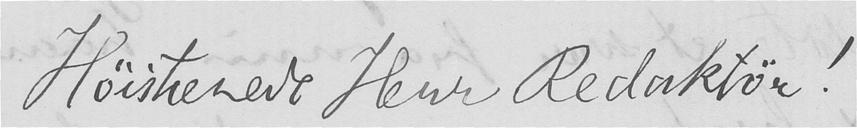Høistærede Herr Redaktør!
